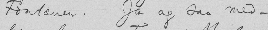Fontænen. Ja og saa med-
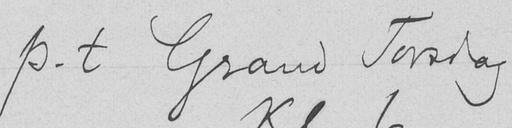p.t Grand Torsdag
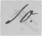10.
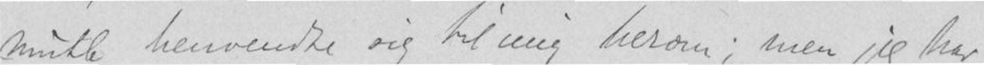muth henvendete sig til mig herom; men jeg har
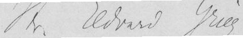Hr. Edvart Grieg
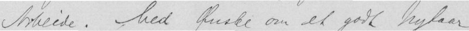Arbeide. hed Ønske om et godt Nytaar
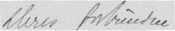Deres forbuedte
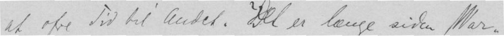at ofre Tid til Andet. Det er længe War-
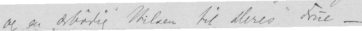og en ærbødig Hilsen til Deres Frue -
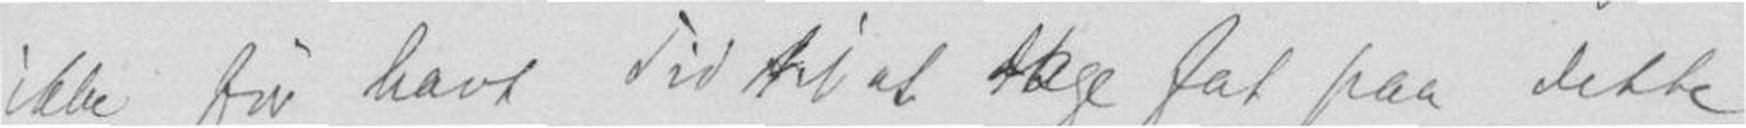ikke før havt Tid til at tøge fat paa dette
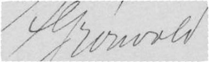A. Grønvold
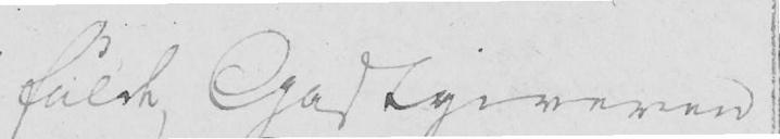fulde, Gastgiveren
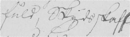fuld, Skydsskaffe
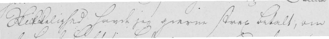Skikkelighed havde jeg gierne strax betalt, om
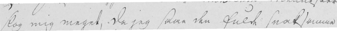slog mig meget, da jeg saae den fulde snaksomme
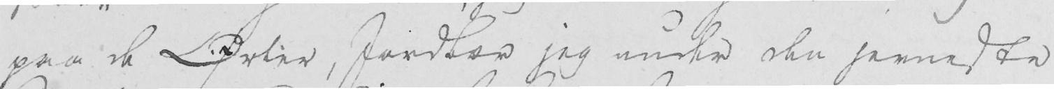paa de Ørter, Jordbær jeg under den jevneste
